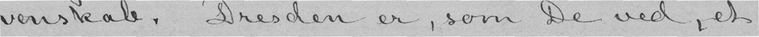venskab. Dresden er, som De ved, et
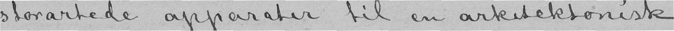storartede apparater til en arkitektonisk
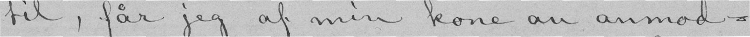til, får jeg af min kone en anmod-
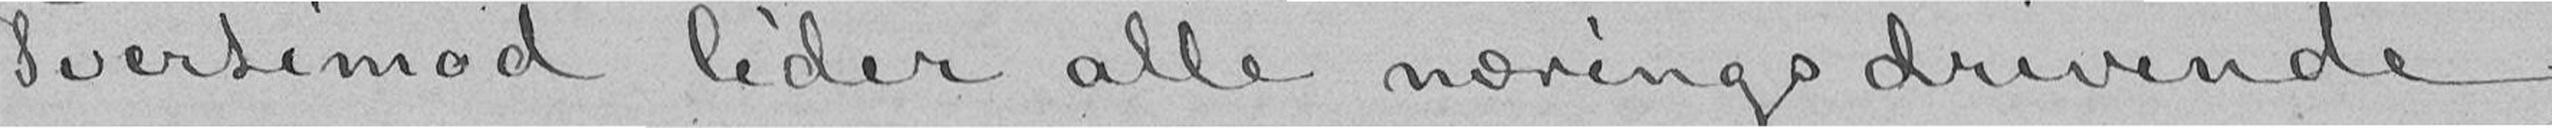Tvertimod lider alle næringsdrivende
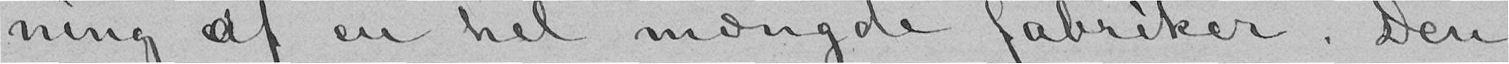ning af en hel mængde fabriker. Den
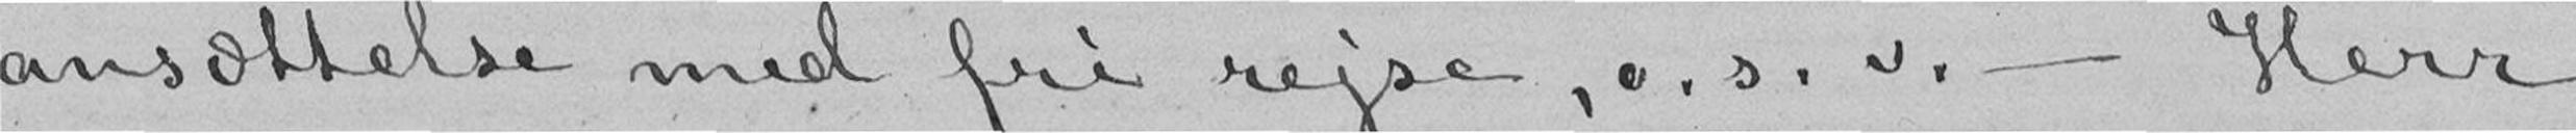ansættelse med fri rejse, o. s. v.- Herr
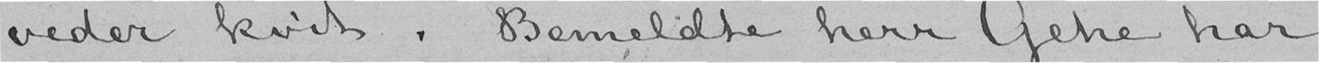veder kvit. Bemeldte herr Gehe har
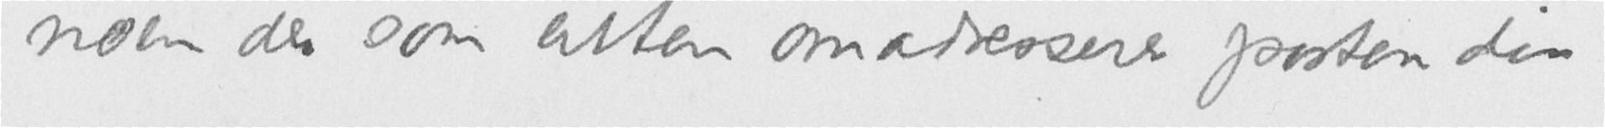noen der som enten omadresserer posten din
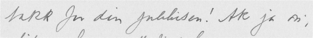takk for din julehilsen! Ak ja du,
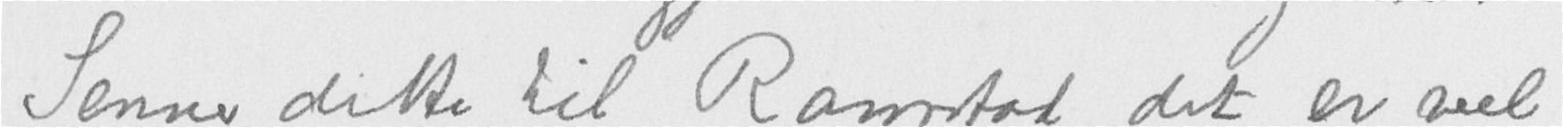Senner dette til Ramstad, det er vel
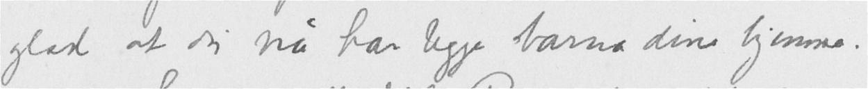glad at du nu har begge barna dine hjemme.
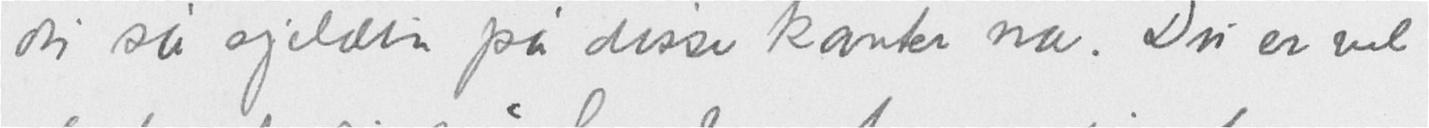du så sjelden på disse kanter nu. Du er vel
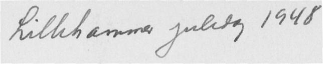Lilletsammer juledag 1948
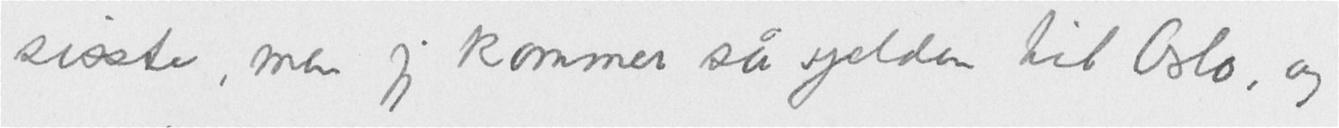sisste, men jeg kommer så sjelden til Oslo, og
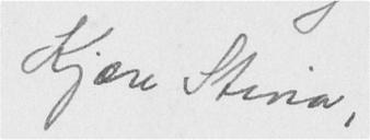Kjære Stina,
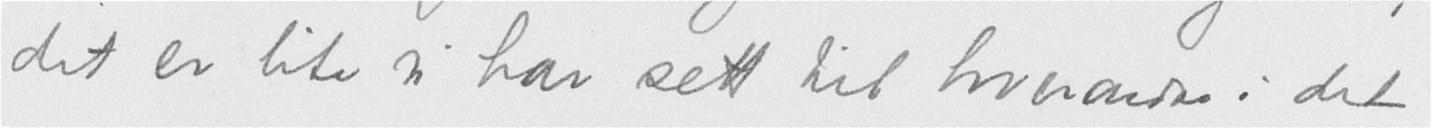det er lite vi har sett til hverandre i det
